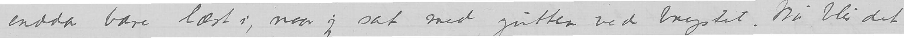endda bare læst i, naar jeg sat med gutten ved brystet. Nu blir det
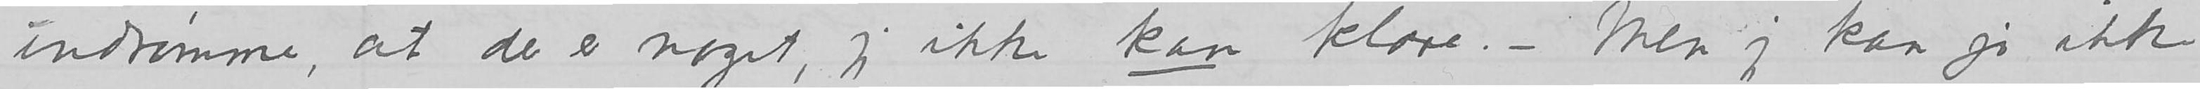indrømme, at der er noget, jeg ikke kan klare. – Men jeg kan jo ikke
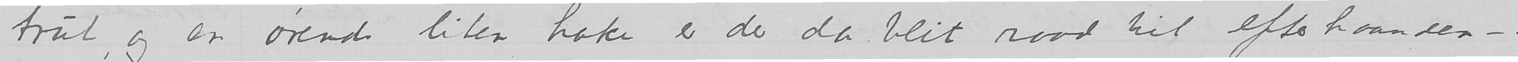trur, og en ørende liten hake er der da blit raad til efterhaanden –.
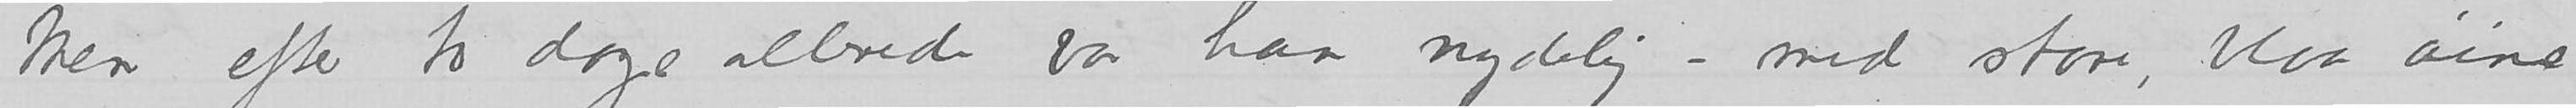Men efter to dager allerede var han nydelig – med store, blaa øine,
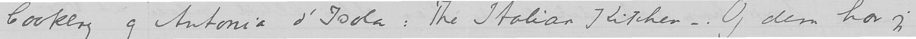Cookery og Antonia d’Isola: The Italian Kitchen –. Og dem har jeg
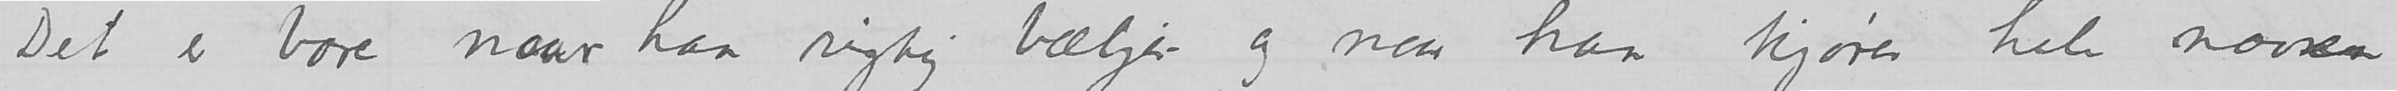Det er bare naar han rigtig bæljer og naar han kjører hele næven
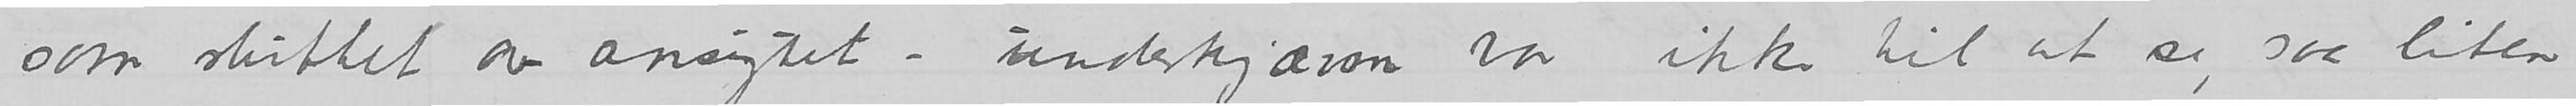som sluttet av ansigtet – underskjæven var ikke til at se, saa liten
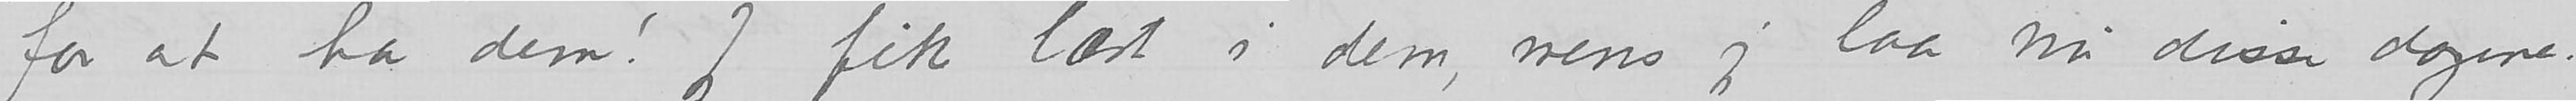for at ha dem! Jeg fik læst i dem, mens jeg laa nu disse dagene.
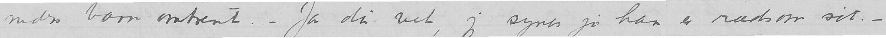neders barn omtrent. – Ja du vet, jeg synes jo han er rædsom søt. –
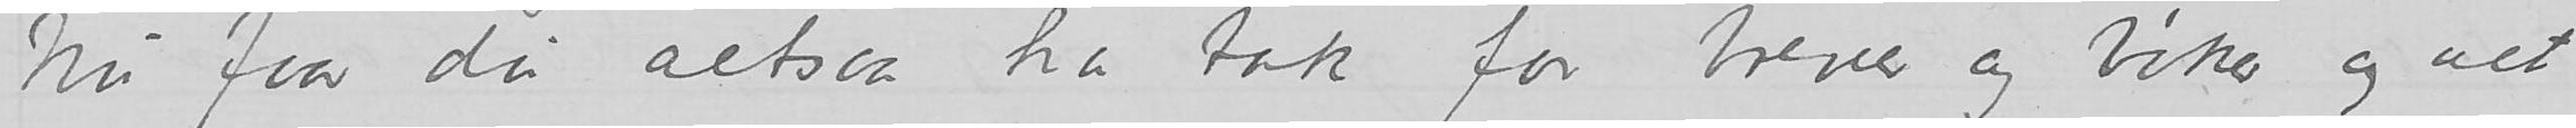Nu faar du altsaa ha tak for brever og bøker og alt
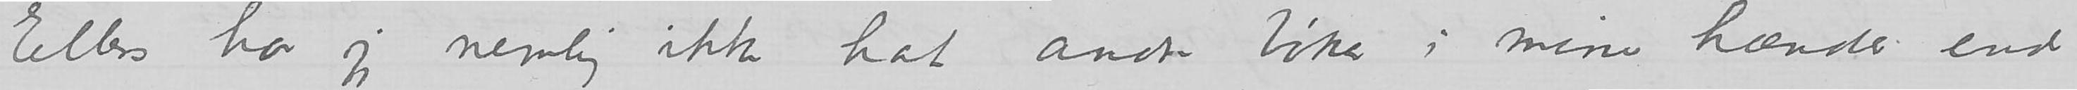Ellers har jeg nemlig ikke hat andre bøker i mine hænder end
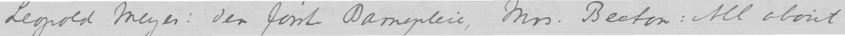Leopold Meyer: Den første Barnepleie, Mrs. Beeton: All about
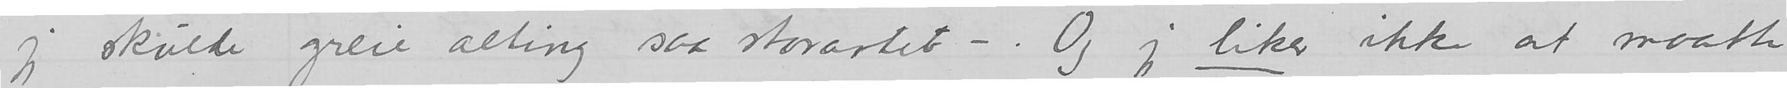jeg skulde greie alting saa stortartet –. Og jeg liker ikke at maatte
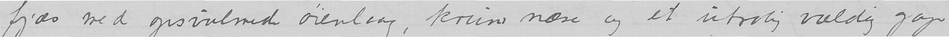fjæs med opsvulmed øienlaag, krum næse og et utrolig vældig gap
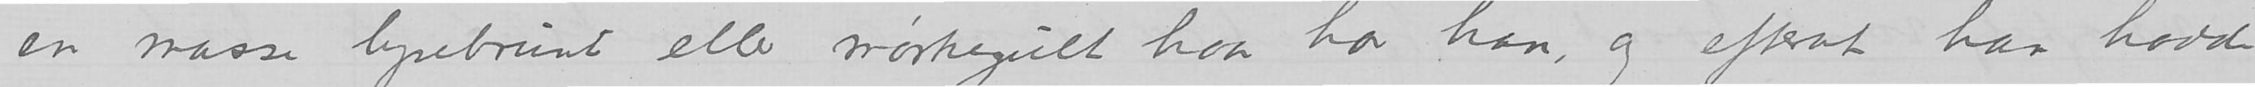en masse lysebrunt eller mørkegult haar har han, og efterat han hadde
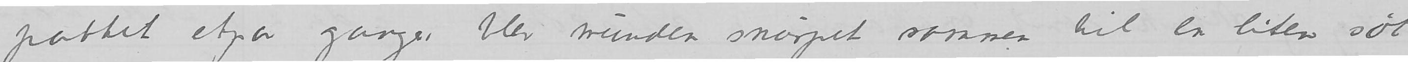pattet etpar ganger blev munden snurpet sammen til en liten søt
